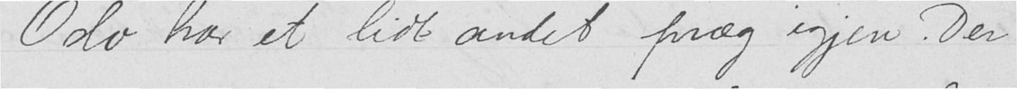Oslo har et lidt andet præg igjen. Der
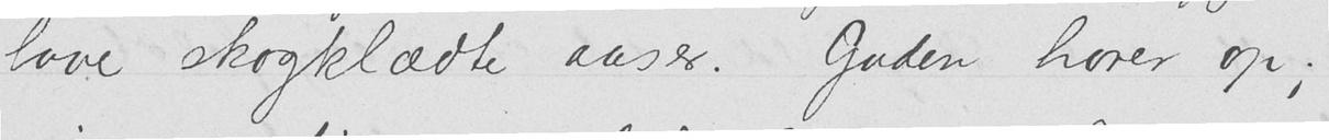lave skogklædte aaser. Gaden hører op;
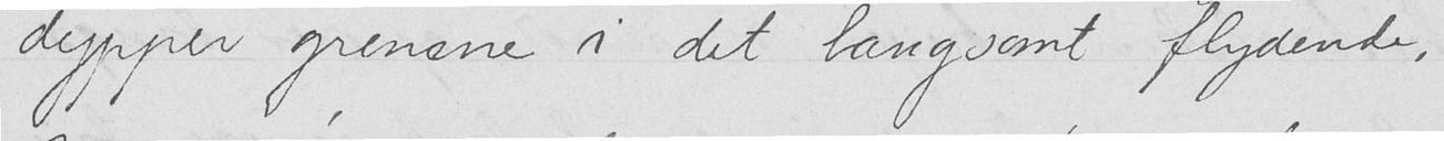dypper grenene i det langsomt flydende,
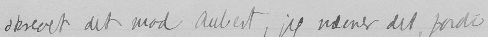skrevet det mod Aubert, jeg nævner det, fordi
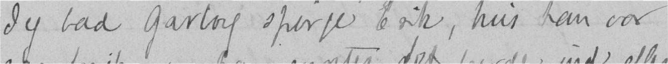Jeg bad Garborg spørge Erik, hvis han var
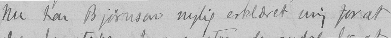Nu har Bjørnson nylig erklæret mig for at
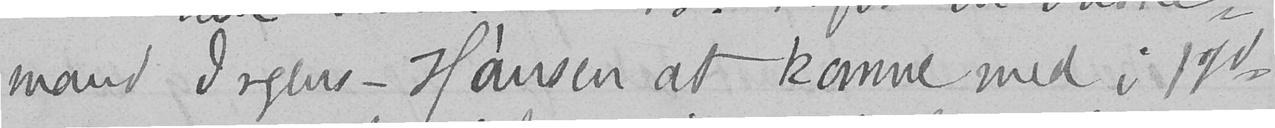mand Irgens-Hansen at komme med i 17d
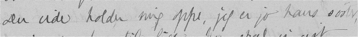Du vide holder mig oppe, jeg er jo hans søster,
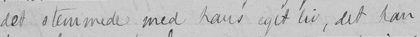det stemmede med hans eget liv, det han
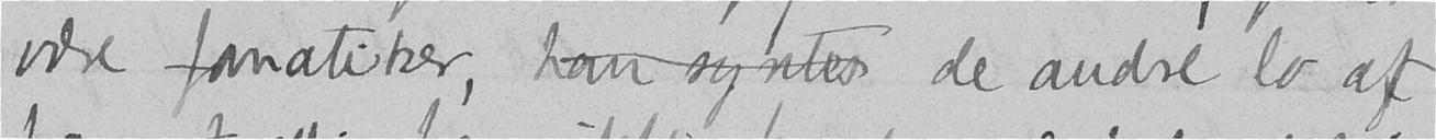være fanatiker, han syntes de andre lo af
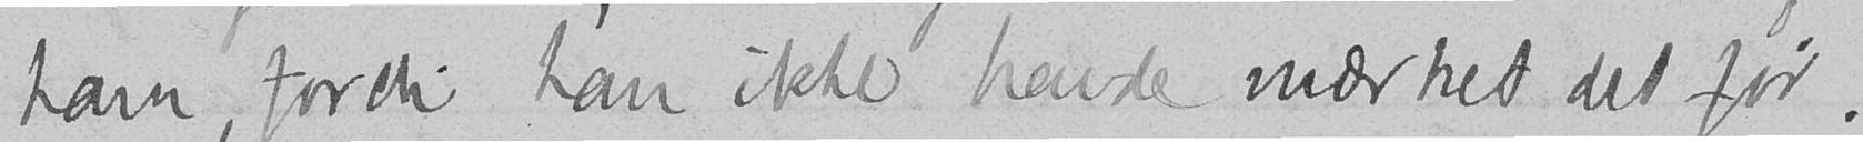ham, fordi han ikke havde mærket det før.
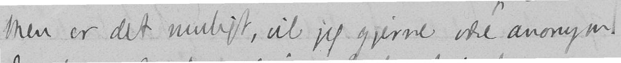Men er det muligt, vil jeg gjerne være anonym.
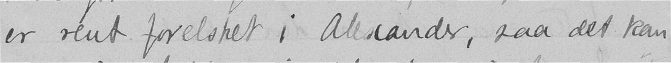er rent forelsket i Alexander, saa det kan
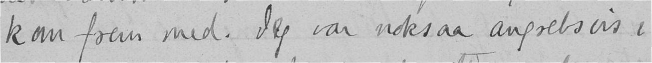kom frem med. Jeg var noksaa angrebsvis i
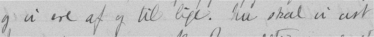og vi ere af og til lige. Nu skal vi vist
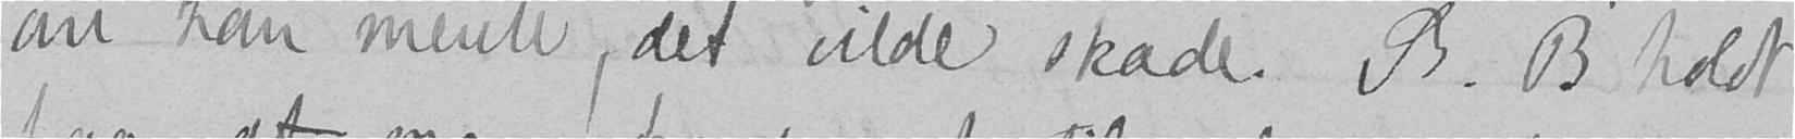om han mente, det vilde skade. B. B holdt
